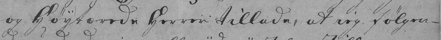og Høytærede herrer tillade, at ieg følgen-
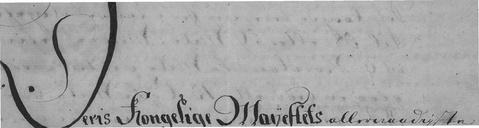Deris Kongelige Majestets allernaadigste
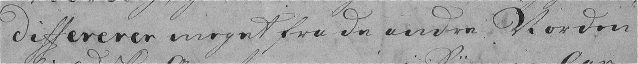differerer meget fra de andre Norden-
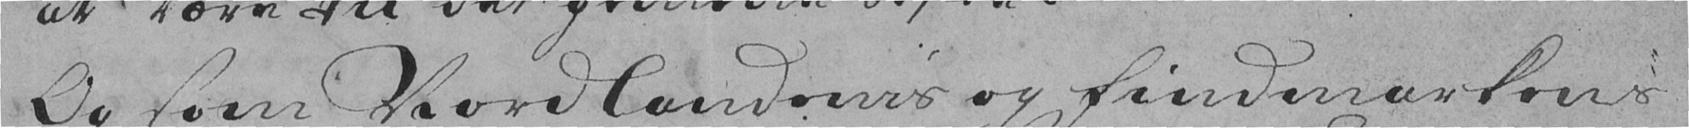Og som Nordlandenis og Findmarkens
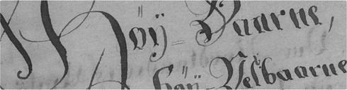Høy-Baarne
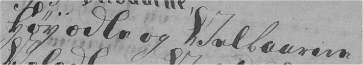Høyædle og Velbaarne
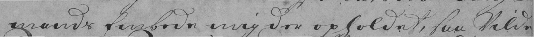mands forbede mig der opholdet, saa vilde
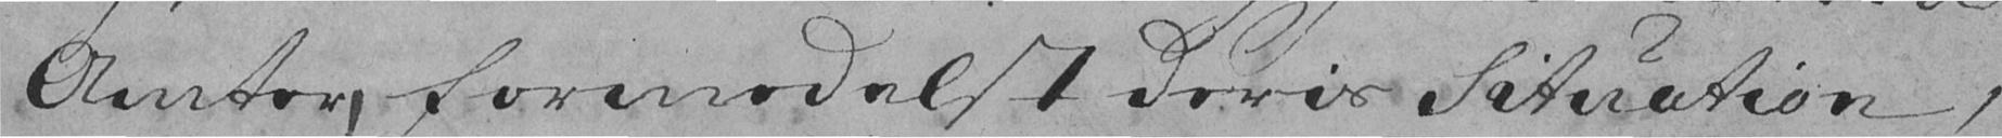Amter, formedelst deris Situation,
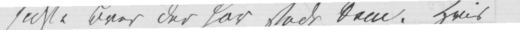sidste Brev der har stødt Dem? Hvis
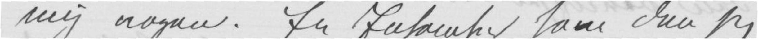mig nogen. En Ensomhed som den jeg
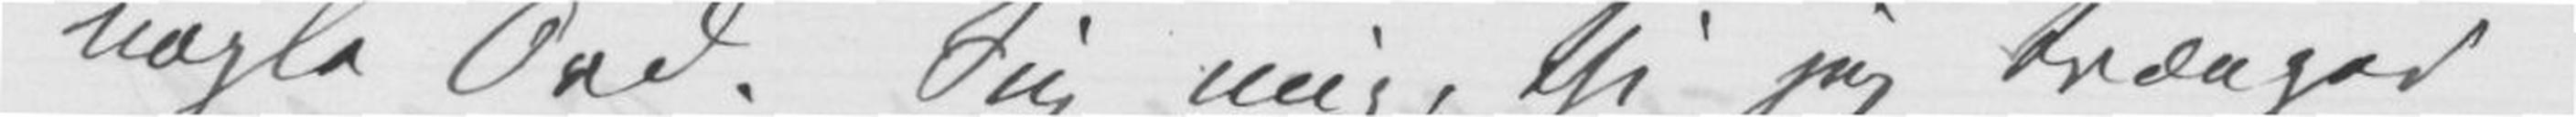nogle Ord. Sig mig, thi jeg trænger
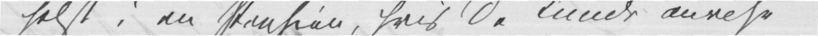helst i en Pension, hvis De kunde anvise
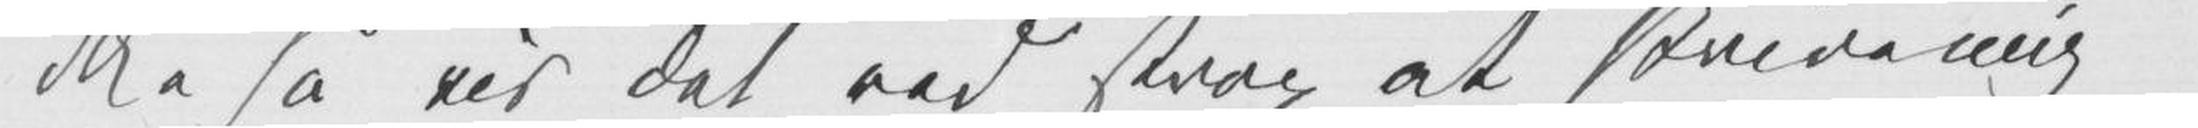ikke så vis det ved strax at skrive mig
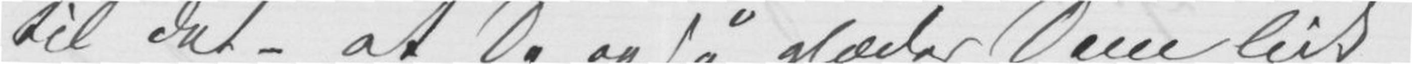til det – at De også glæder Dem lidt
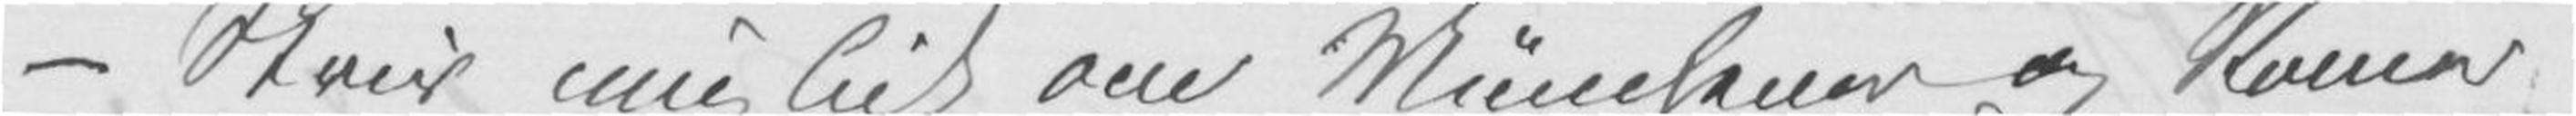– Skriv mig lidt om Münchener og Romer=
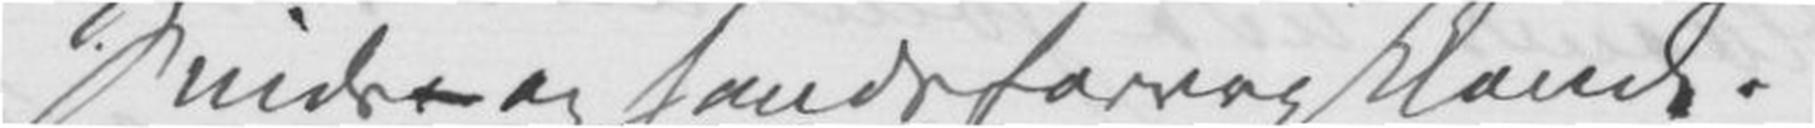Sinds- og sands forrykkende.
